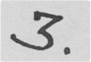3.
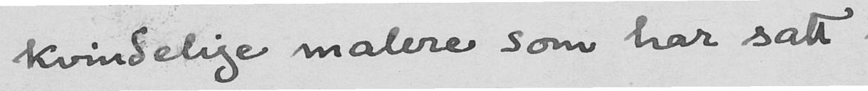kvindelige malere som har satt
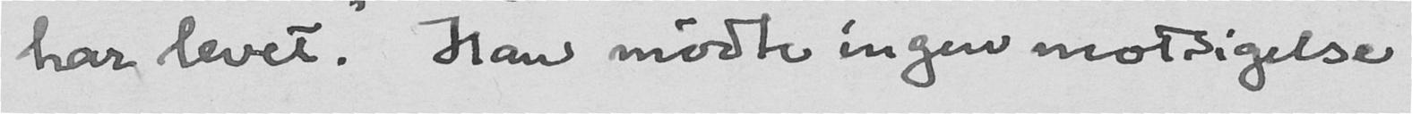har levet." Han mødte ingen motsigelse
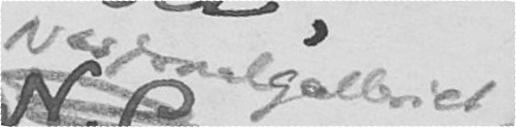Nasjonalgalleriet
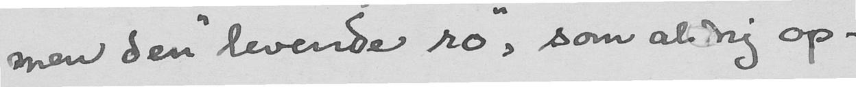men den "levende ro", som aldrig op-
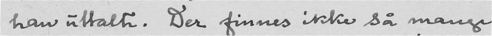han uttalte. Der finnes ikke så mange
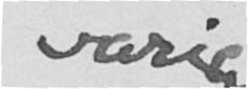varig
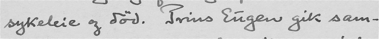sykeleie og død. Prins Eugen gik sam-
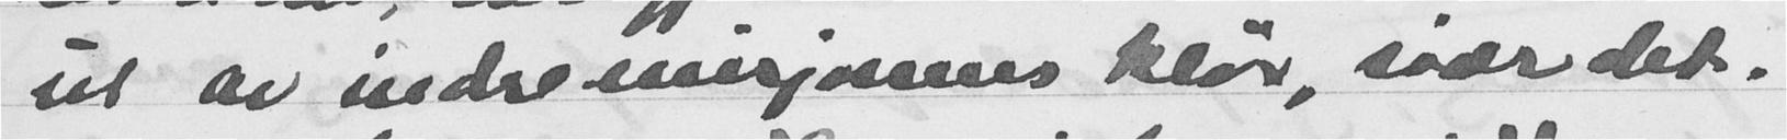ut av indremisjonens klør, sies det.
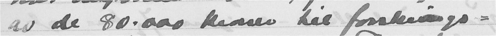av de 80.000 kroner til forsknings-
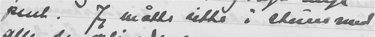pent. Jeg måtte sitte i stuen med
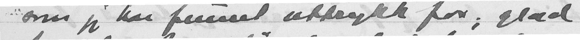som jeg har funnet uttrykk for; glad
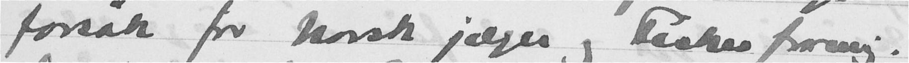forsøk for Norsk jæger og Fisker forening.
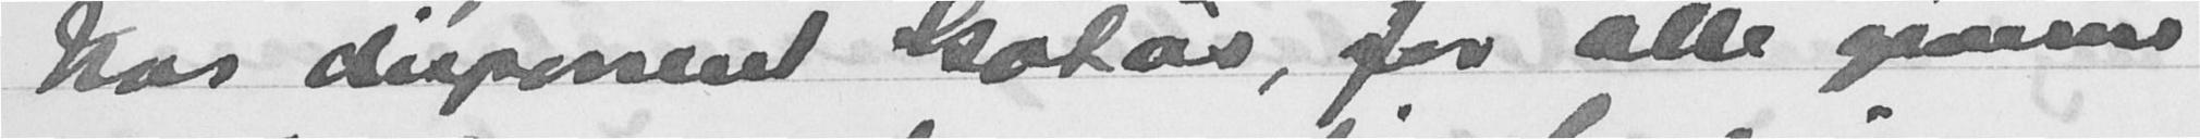hos disponent Haukås, for alle giverne
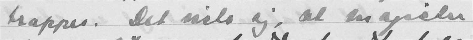trapper. Det viste sig, at magister
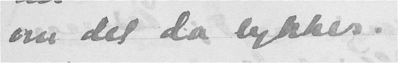om det da lykkes.
